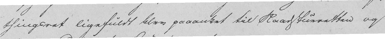thingsret ligefuldt blev paaanket til Raadstueretten og
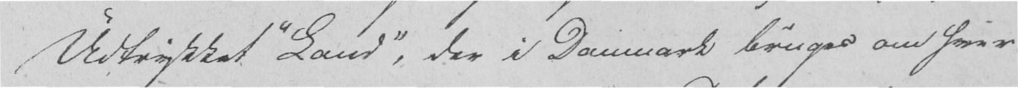Udtrykket "Land", der i Danmark bruges om hver
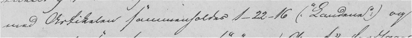med Artikelen sammenholdes 1-22-16 (: "Landene" :) og
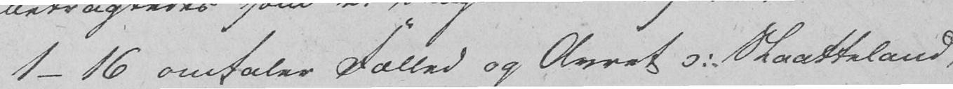1-16 omtaler Fælled og Avret s. Slaatteland,
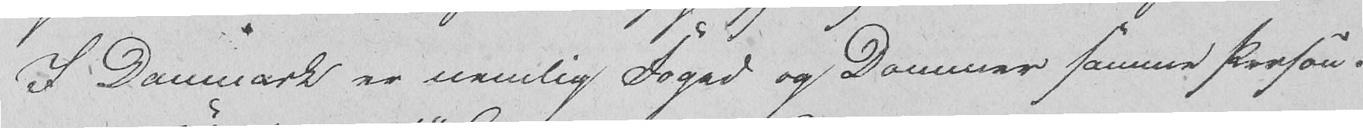I Danmark er nemlig Foged og Dommer samme Person.
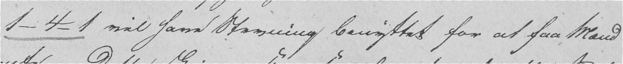1-4-1 vil have Stevning benyttet for at faa Mænd
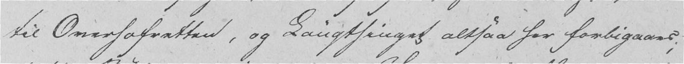til Overhofretten, og Laugthinget altsaa her forbigaaes;
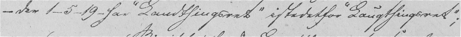- der 1-5-19 - har "Landthingsret" istedetfor "Laugthingsret";
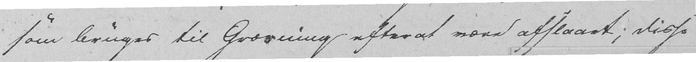som bringes til Græsning efterat være afslaaet; disse
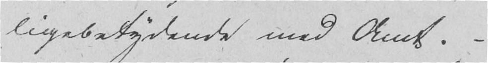ligebetydende med Amt. -
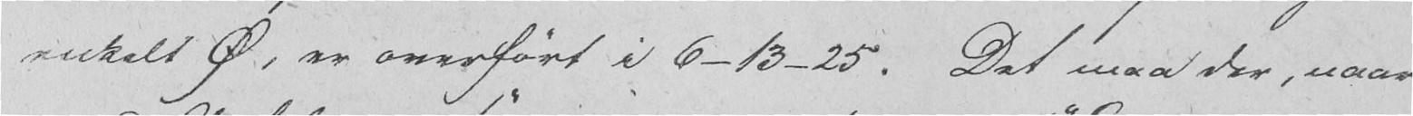enkelt Ø, er overført i 6-13-25. Det maa der, naar
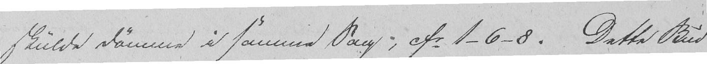skulde dømme i samme Sag, cfr 1-6-8. Dette Bud
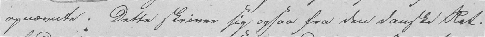opnævnte. Dette skriver sig ogsaa fra den danske Ret.
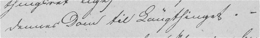dennes Dom til Laugthinget. -
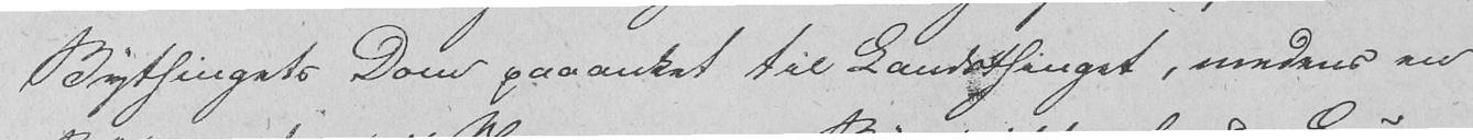Bythingets Dom paaanket til Landsthinget, medens en
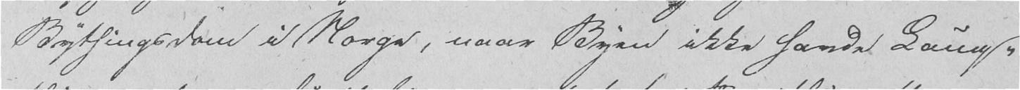Bythingsdom i Norge, naar Byen ikke havde Laug-
